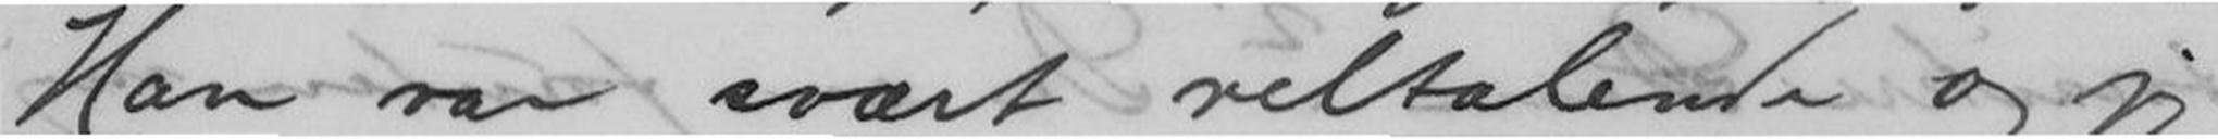Han var svært veltalende og jeg
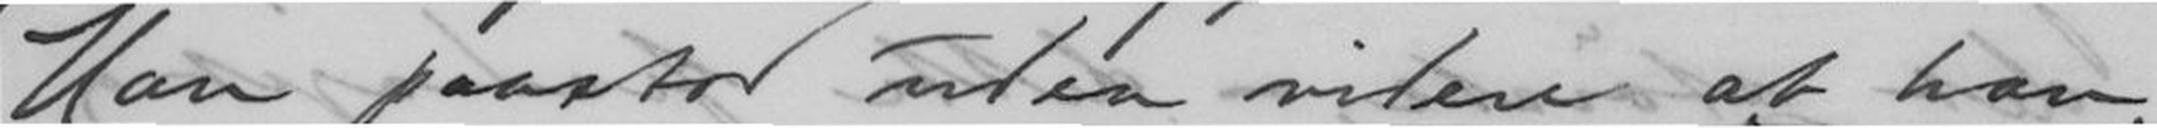Han paastod uden videre at han,
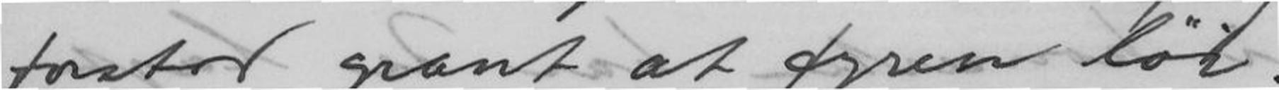forstod grant at fyren løi!
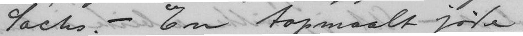Sachs. - En topmaalt jøde
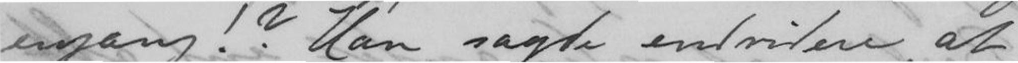engang!? Han sagde endvidere, at
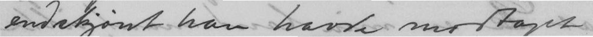enskjønt han havde modtaget
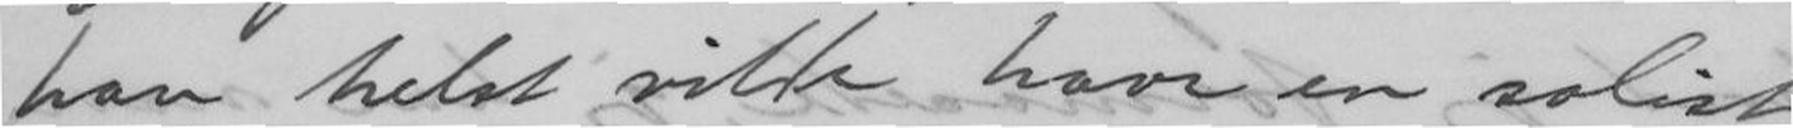han helst vilde have en solist
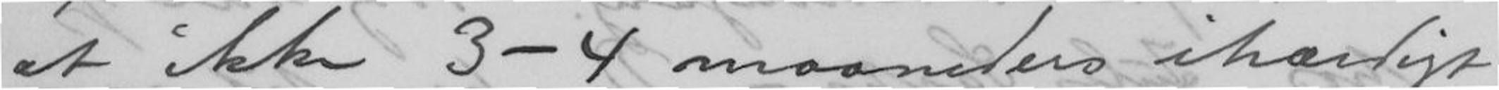at ikke 3-4 maaneders ihærdigt
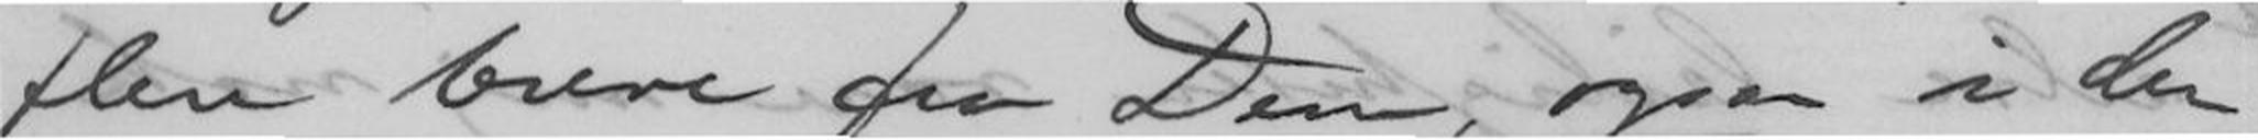flere breve fra Dem, ogsaa i den
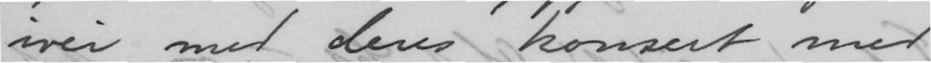ivei med deres konsert med
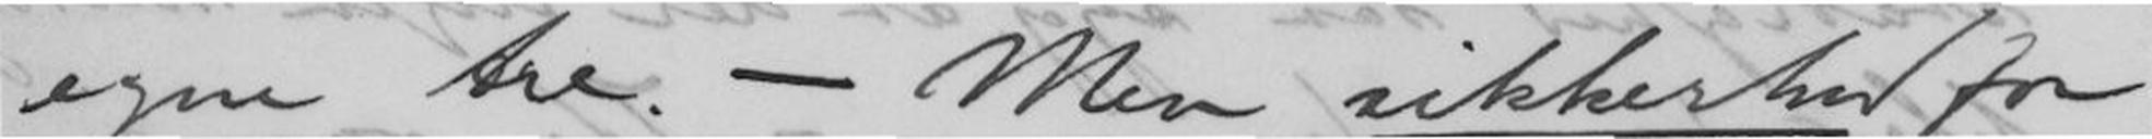egne tre. - Men sikkerhed for
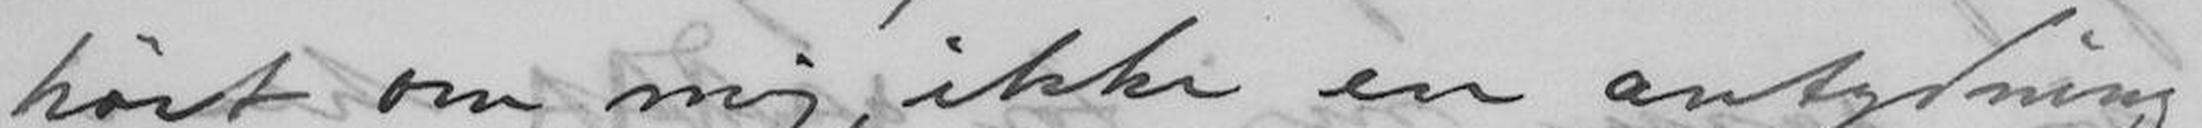hørt om mig, ikke en antydning
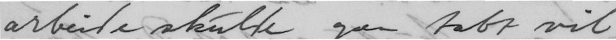arbeide skulde gaa tabt vil
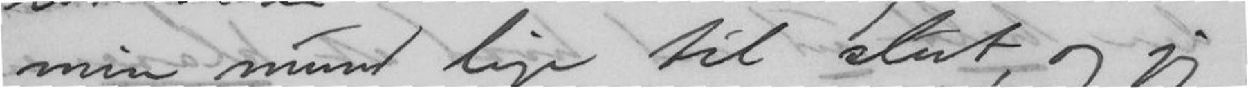min mund lige til slut og jeg
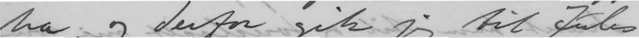ha, og derfor gik jeg til Jules
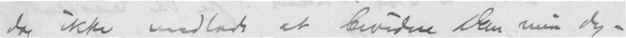dog ikke indlade at bevidne Dem min dy-
